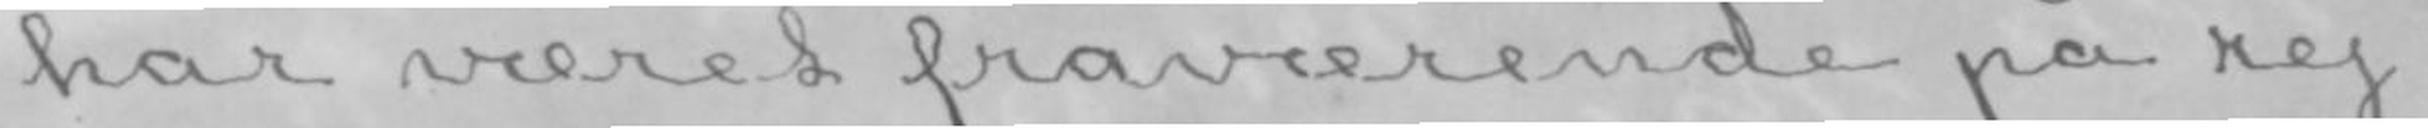har været fraværende på rej-
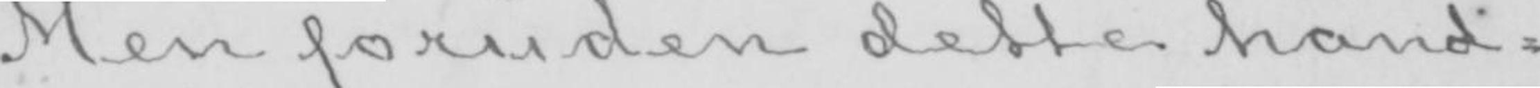Men foruden dette hand-
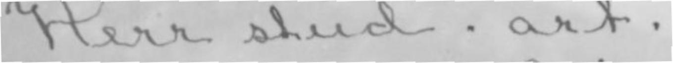Herr stud. art.
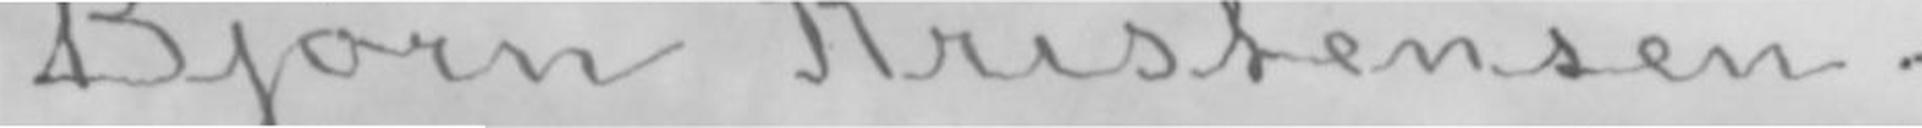Bjørn Kristensen.
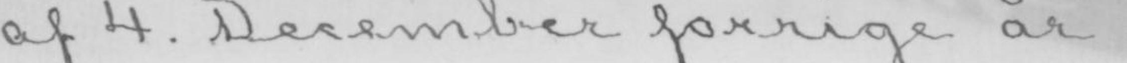af 4. December forrige år.
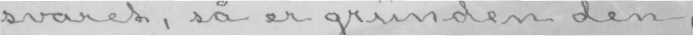svaret, så er grunden den,
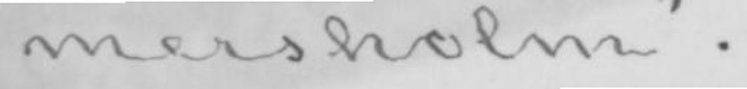mersholm».
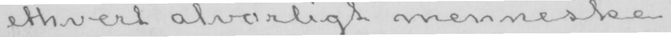ethvert alvorligt menneske
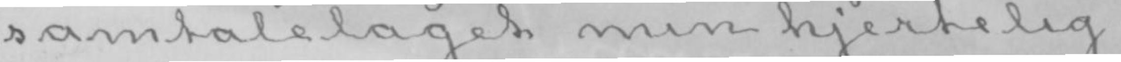samtalelaget min hjertelig-
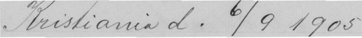Kristiania d. 6/9 1905.
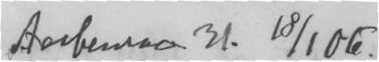Aabenraa 31. 18/1 06.
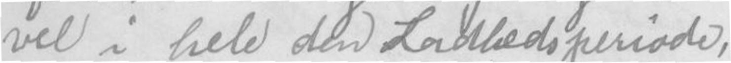vel i hele den Ladhedsperiode,
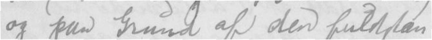og paa Grund af den fuldstæn-
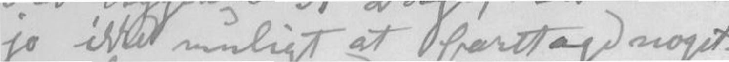jo ikke muligt at foretage noget
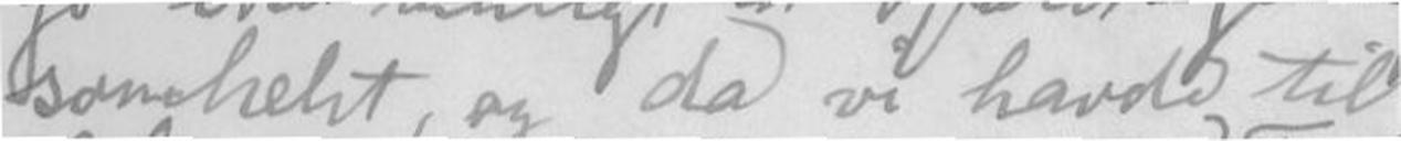somhelst, og da vi havde til
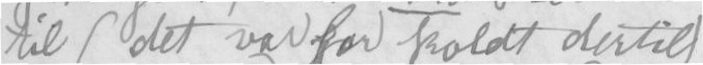til (det var for koldt dertil)
